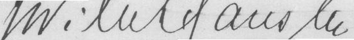Wilhelm Hansen
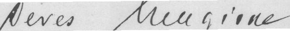Deres hengivne
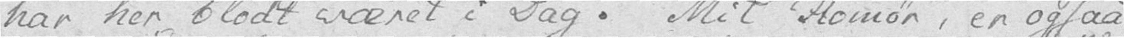har her blodt været i Dag. Mit Homør, er ogsaa
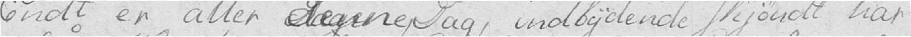Endt er atter denne Dag, indbydende skjøndt har
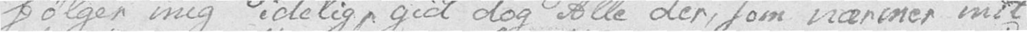følger mig idelig, gid dog Alle der, som nærmer mit
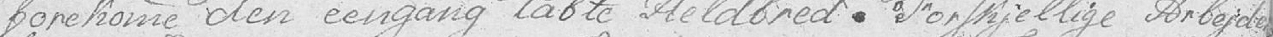forekomme den eengang tabte Heldbred. Forskjellige Arbejde
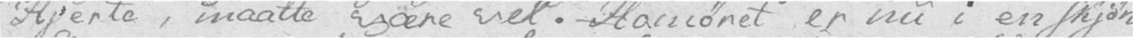Hjerte, maatte være vel. Homøret er nu i en skjøn
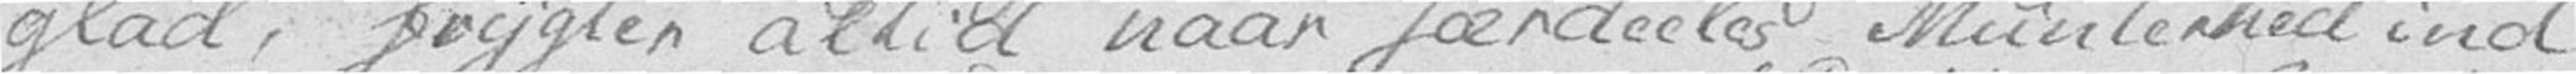glad, frygter altid naar særdeeles Munterhed ind
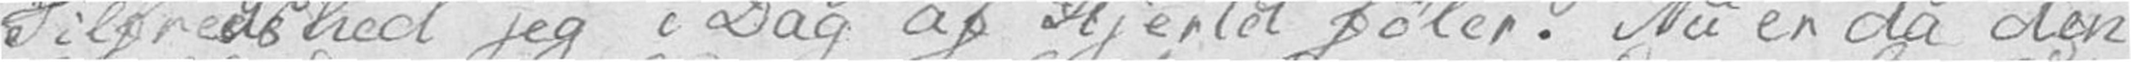Tilfredshed jeg i Dag af Hjertet føler. Nu er da den
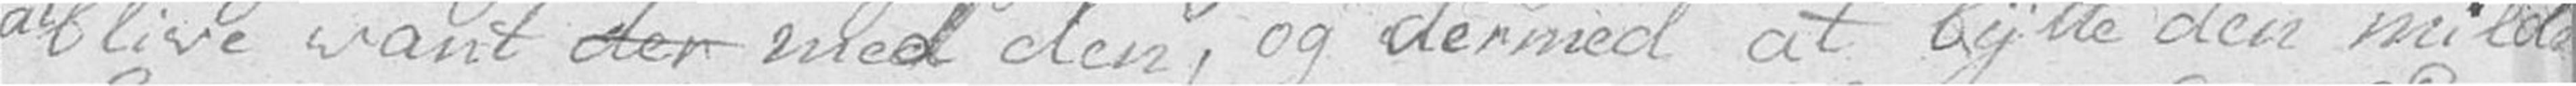at blive vant den med den, og dermed at bytte den milde
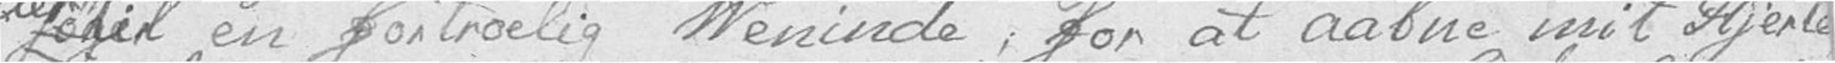iler fortil en fortroelig Veninde, for at aabne mit Hjerte
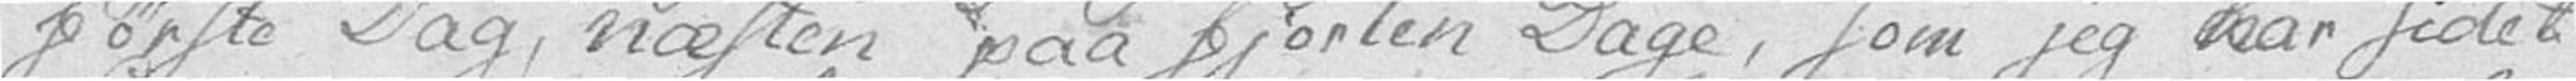første Dag, næsten paa fjorten Dage, som jeg har sidet
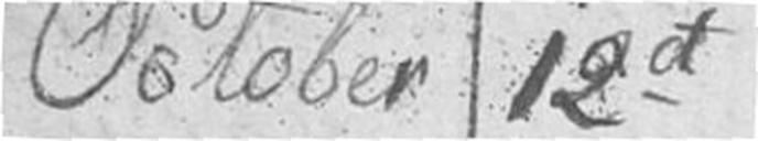October 12d
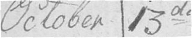October 13de
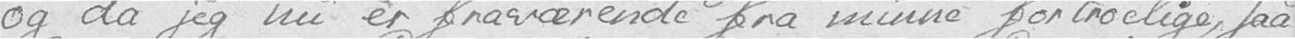og da jeg nu er fraværende fra minne fortroelige, saa
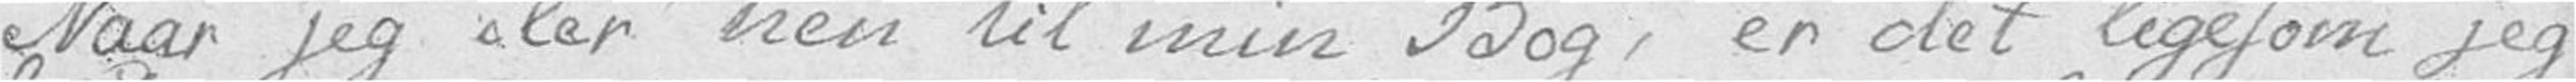Naar jeg iler hen til min Bog, er det ligesom jeg
